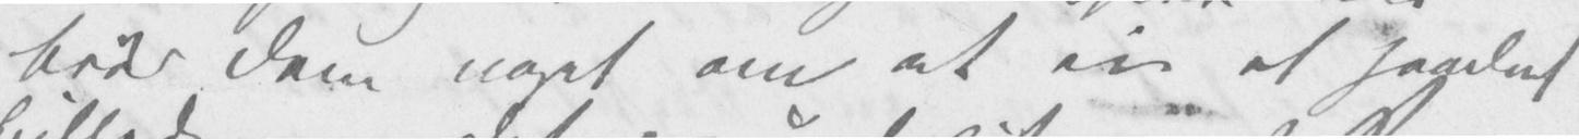brød Dem noget om at eie et saadant
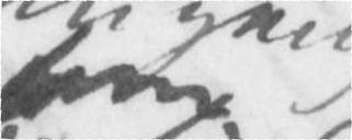<...>
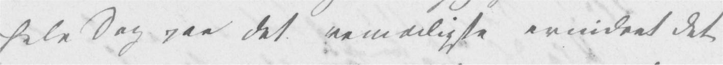hele Dag paa det vemodigste erindret det.
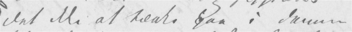det ikke at tænke paa i denne
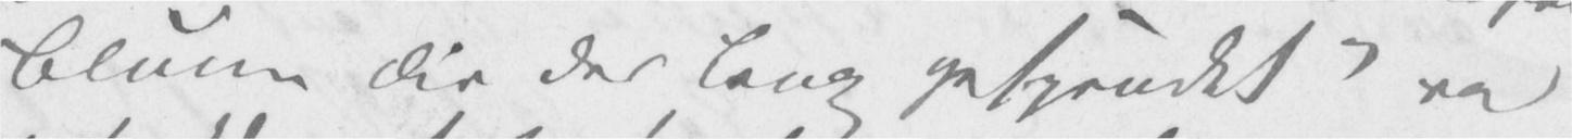Blumen die der Lenz gespendet» var
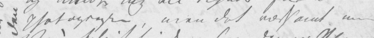photograph, men det rædsomt
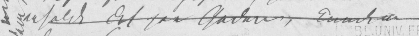anholde det paa Gaden, kunde man
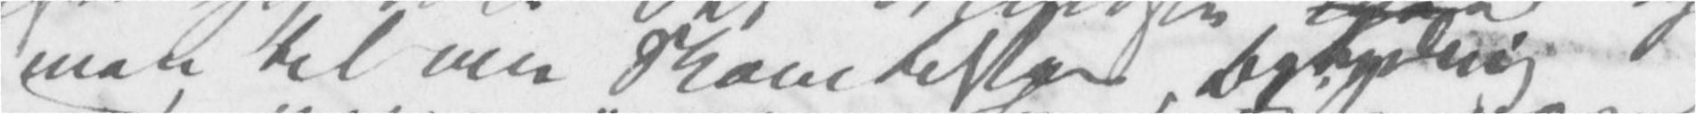maa til min Skam tilstaae Betydning
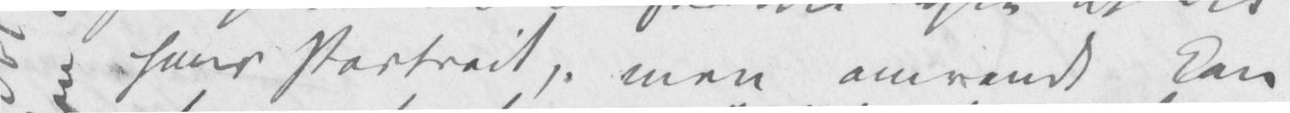hans Portrait, men omvendt kan
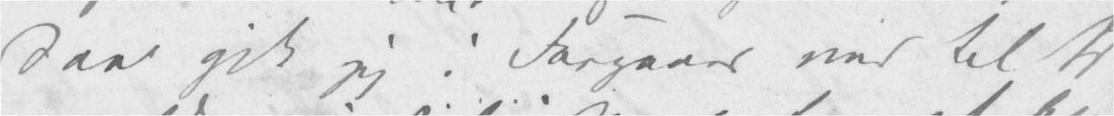Saa gik jeg i Forgaars ned til W
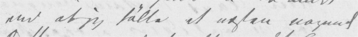end at jeg følte et næsten nagende
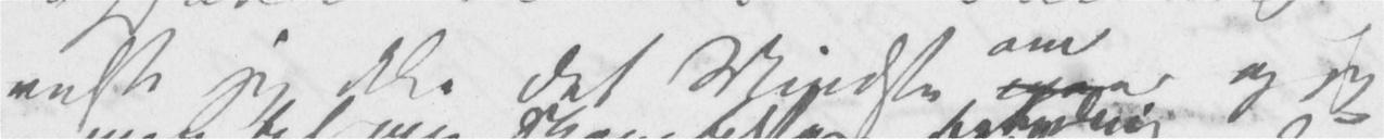vidste jeg ikke det Mindste igaar og jeg
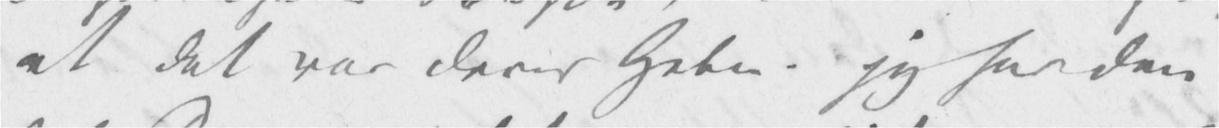at det var Deres Gebu. jeg har den
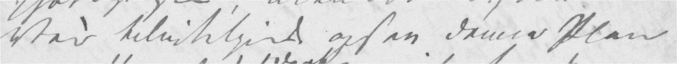Veir tilintetgjorde ogsaa denne Plan
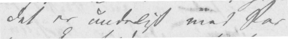Det er underligt med Por-
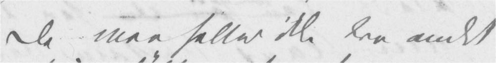De maa heller ikke tro andet
